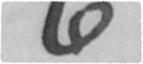6
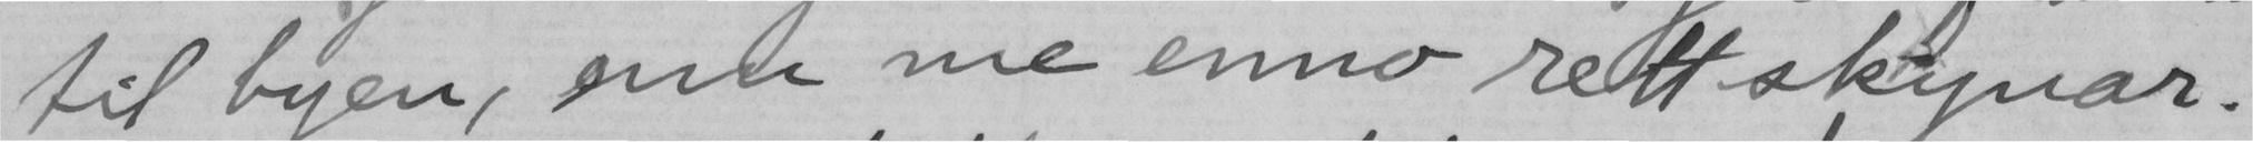til byen, enn me enno rett skynar.
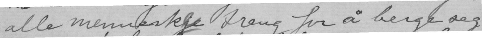alle menneskje treng for å berge seg
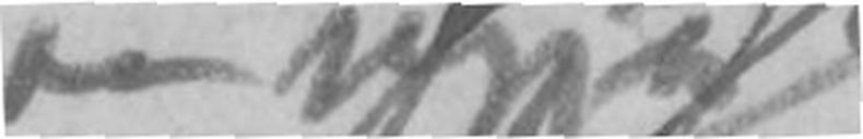nytte
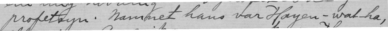profetsyn. Namnet hans var Hayen-wat-ha,
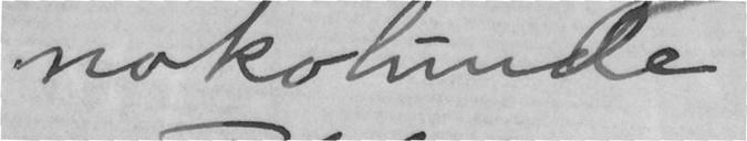nokolunde
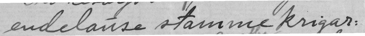endelause stammekrigar:
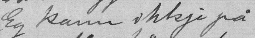Eg kann ikkje på
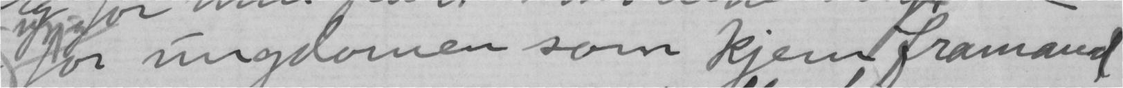for ungdomen som kjem framand
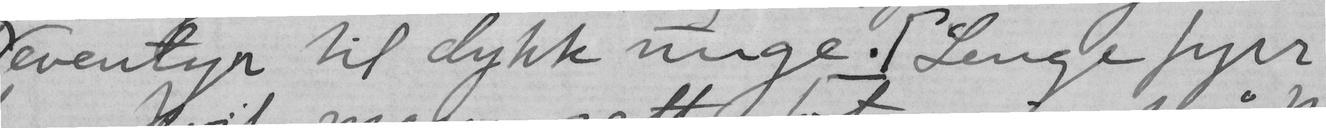eventyr til dykk unge. Lenge fyrr
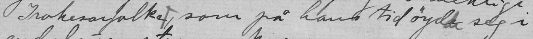Irokesarfolket, som på hans tid øyde seg i
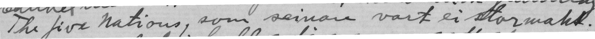The five Nations, som seinare vart ei stormakt.
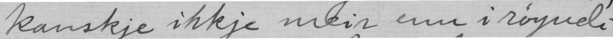kanskje ikkje meir enn i røyndi
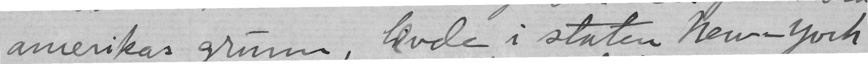amerikas grunn, levde i staten New-York
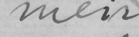meir
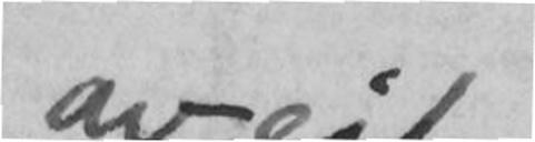av eit
